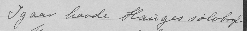Igaar havde Hauges sølvbryl-
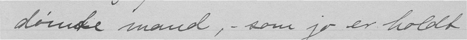dømte mand, - som jo er holdt
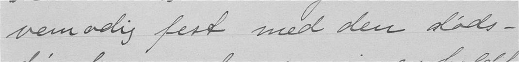vemodig fest med den døds-
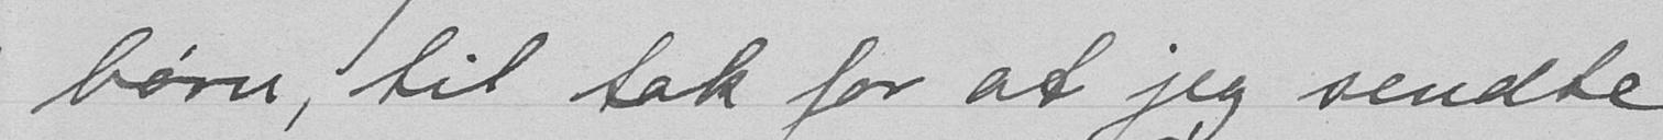børn, til tak for at jeg sendte
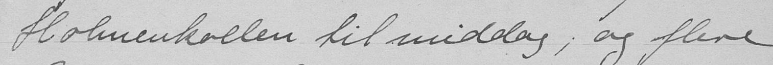Holmenkollen til middag, og flere
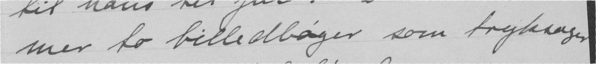mer to billedbøger som tryksager
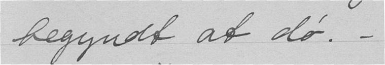begyndt at dø. -
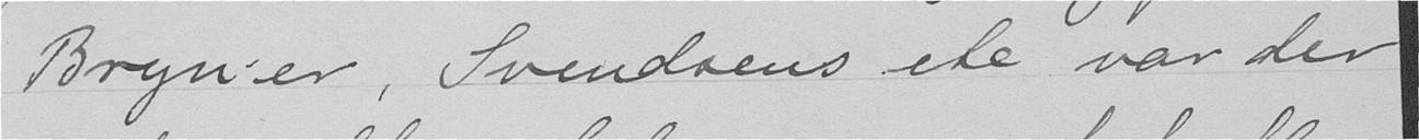Bryn'er, Svendsens ete var der
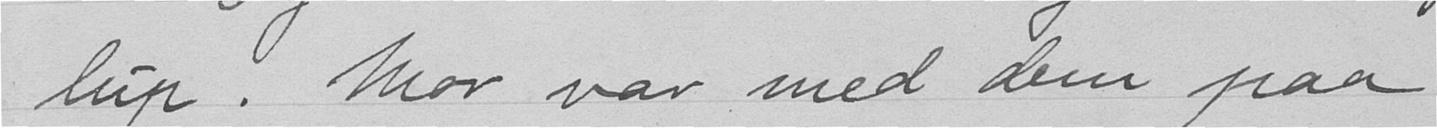lup. Mor var med dem paa
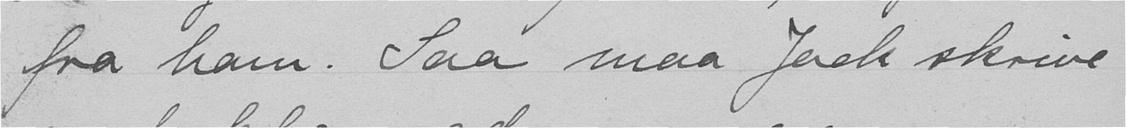fra ham. Saa maa Jack skrive
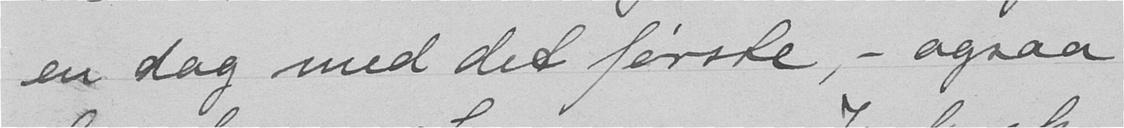en dag med det første, - ogsaa
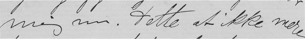mig nu. Dette at ikke mere
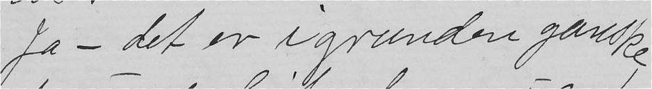Ja - det er igrunden ganske
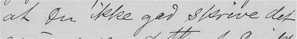at Du ikke gad skrive det
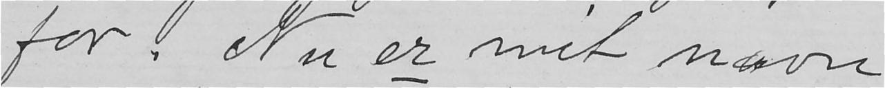for. Nu er mit navn
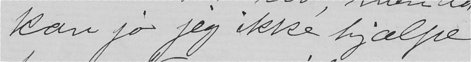kan jo jeg ikke hjælpe
